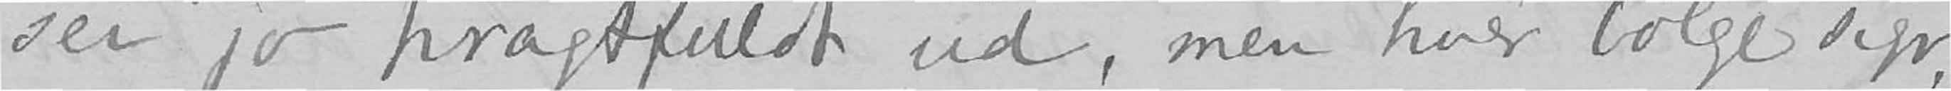ser jo pragtfuldt ud, men hver bølge siger,
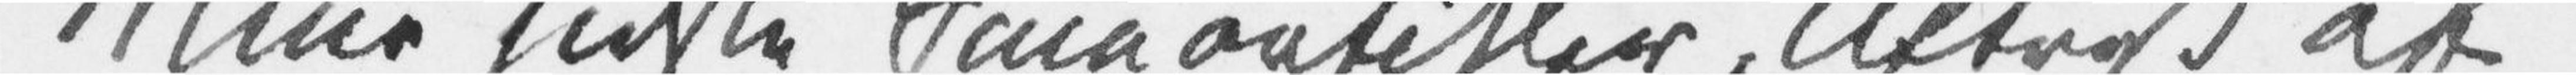Mine sidste Småartikler, Aftryk af
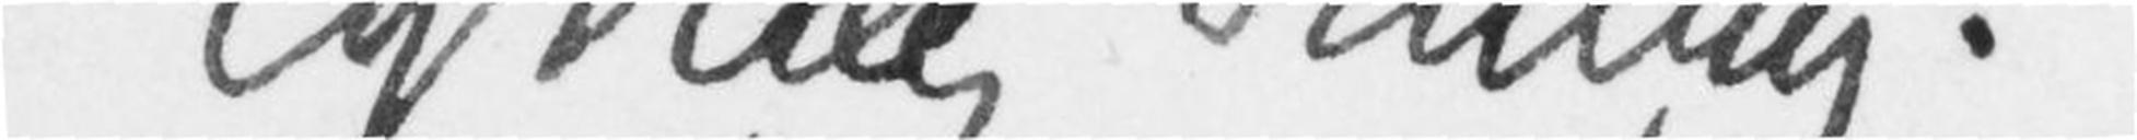lykkelig Stilling».
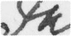de
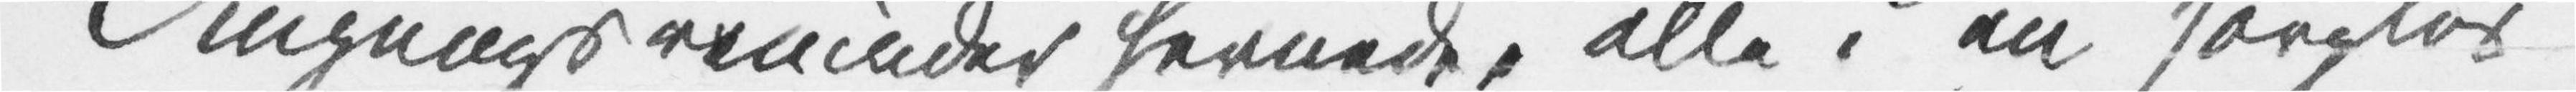Omgangsveninder hernede, alle i en «sorgløs
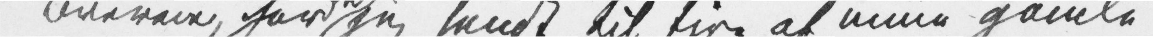Brevene, hav jeg sendt til fire af mine gamle
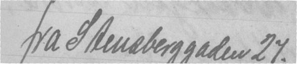Fra Sensberggaden 27.
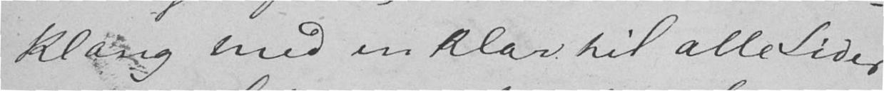klang med en klar til alle Sider
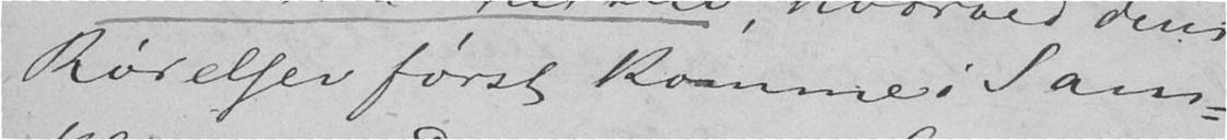Rørelser først kommer i Sam-
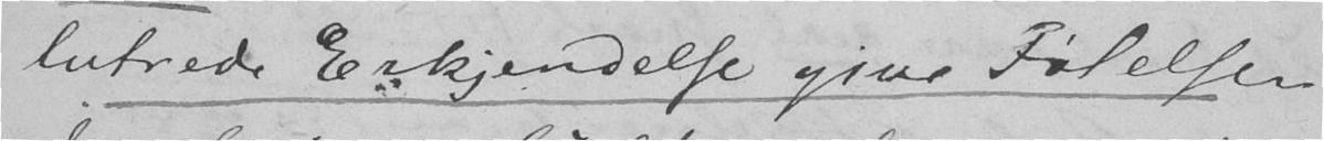lutrede Erkjendelse give Følelsen
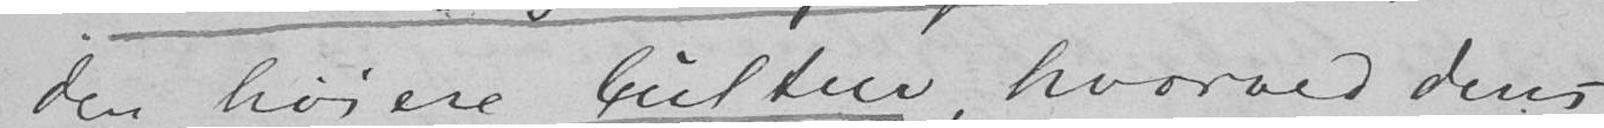den høiere Cultur, hvorved dens
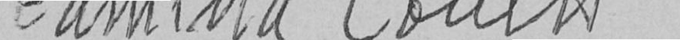Camilla Collett
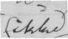ikke
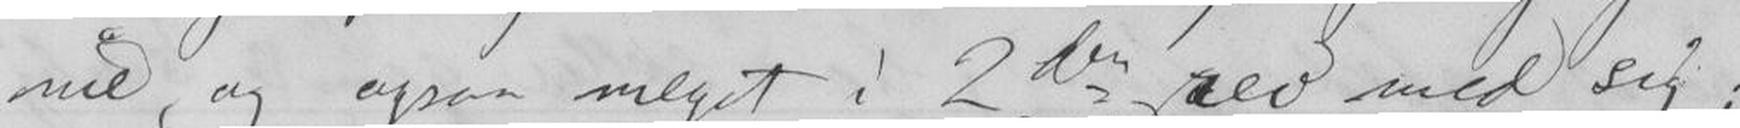nie, og ogsaa meget i 2den rev med sig;
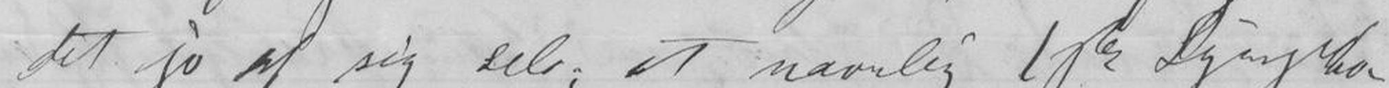det jo af sig selv; at navnlig 1ste Sympho-
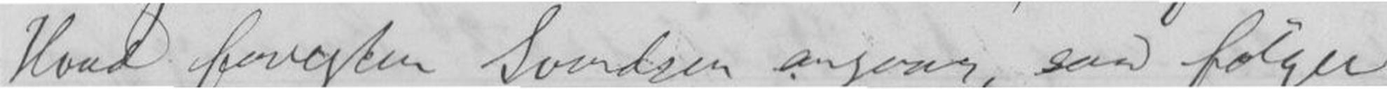Hvad forresten Svendsen angaar, saa følger
